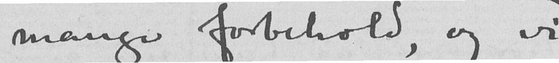mange forbehold, og vi
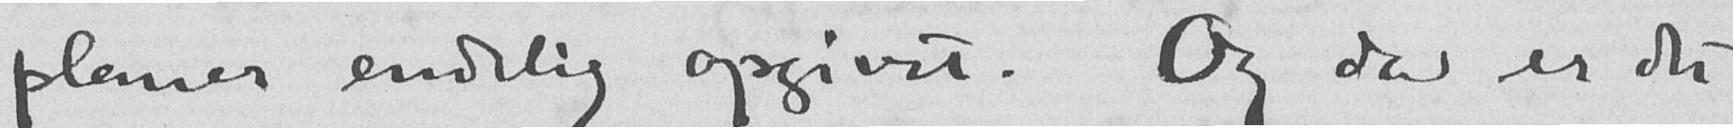planer endelig opgivet. Og da er det
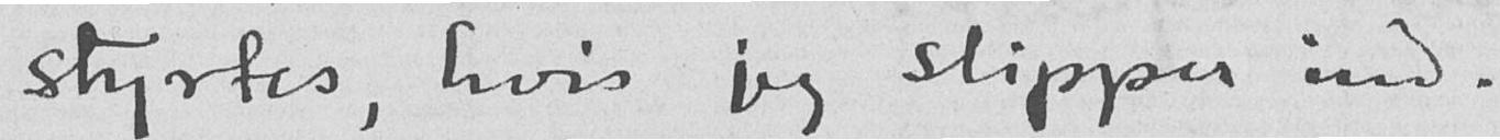styrtes, hvis jeg slipper ind.
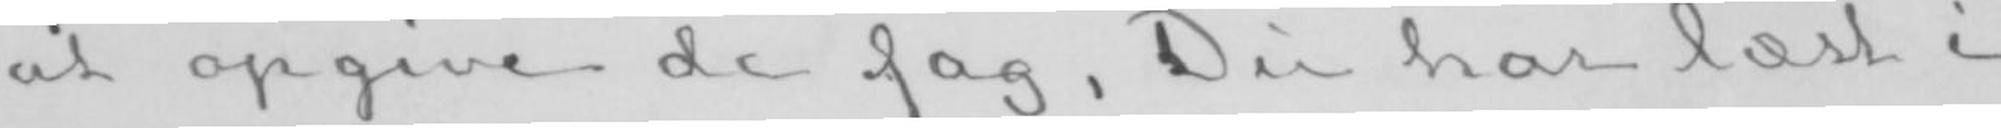at opgive de fag, Du har læst i
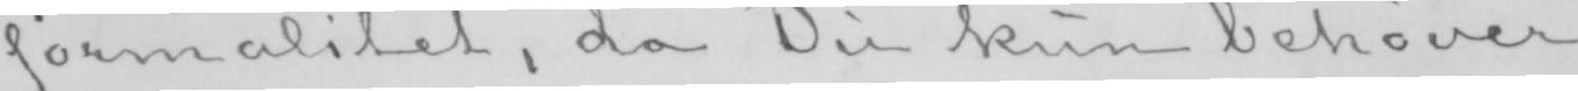formalitet, da Du kun behøver
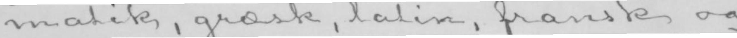matik, græsk, latin, fransk og
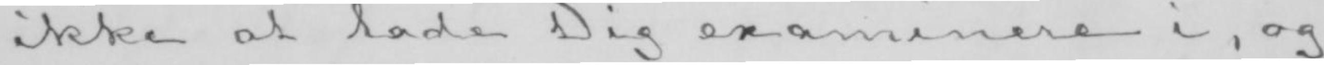ikke at lade Dig examinere i, og
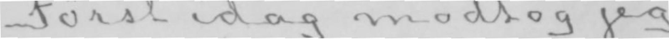Først idag modtog jeg
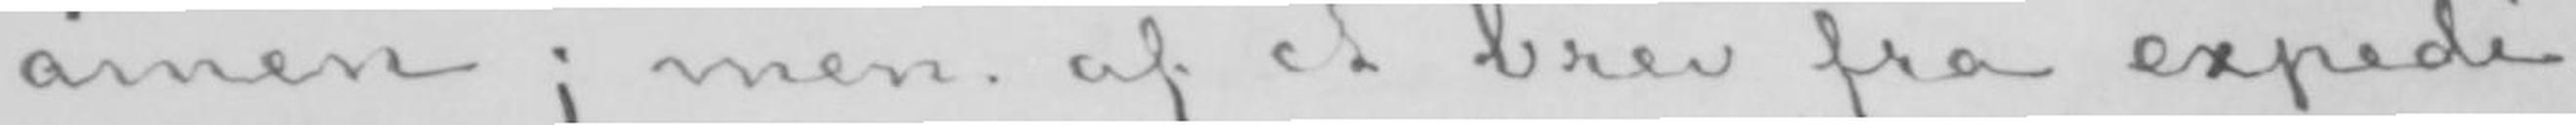amen; men af et brev fra expedi-
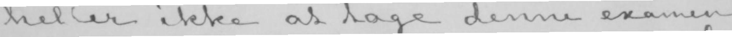heller ikke at tage denne examen
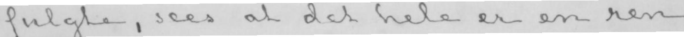fulgte, sees at det hele er en ren
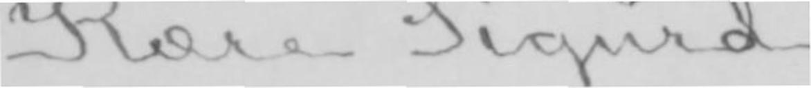Kære Sigurd!
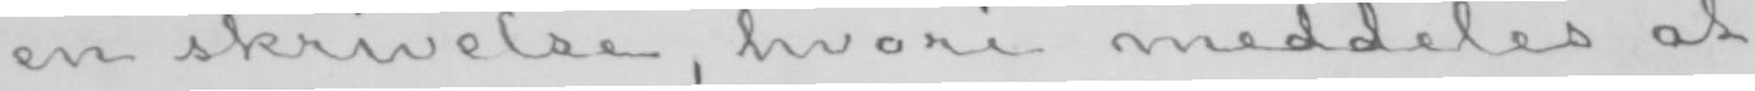en skrivelse, hvori meddeles at
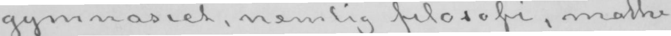gymnasiet, nemlig filosofi, mathe-
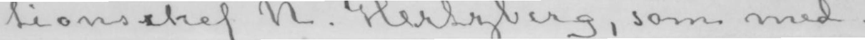tionsschef N. Hertzberg, som med-
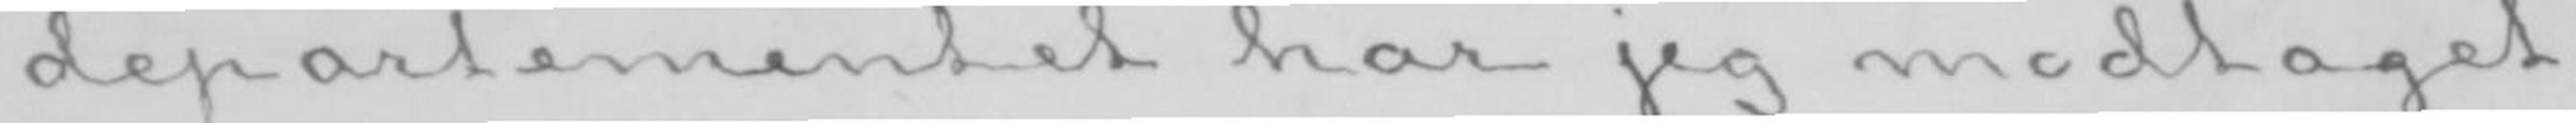departementet har jeg modtaget
